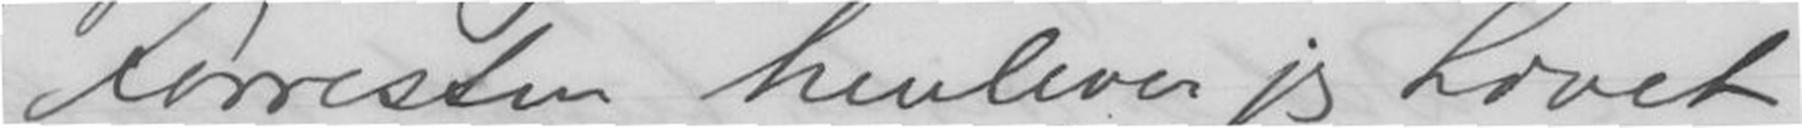Forresten henlever jeg Livet
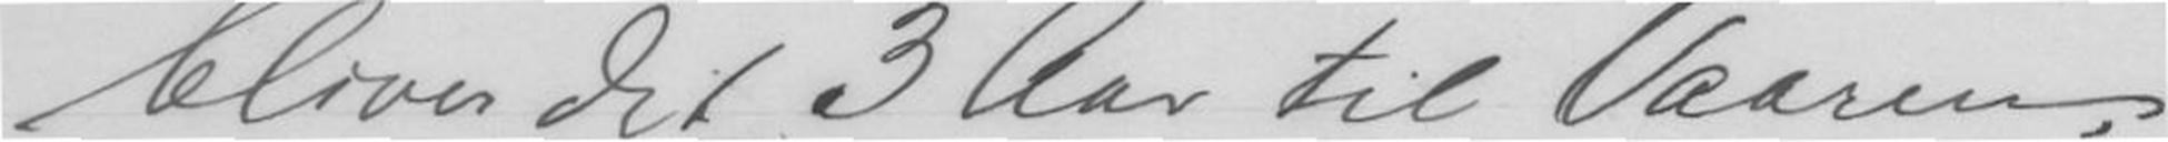bliver det 3 Aar til Vaaren.
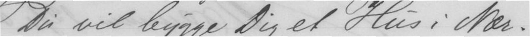Du vil bygge Dig et Hus i Nær-
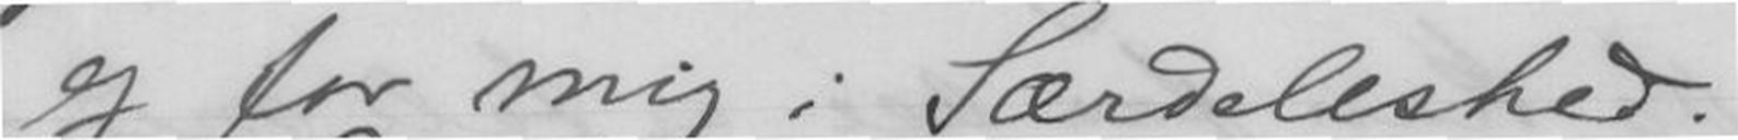og for mig i Særdeleshed.
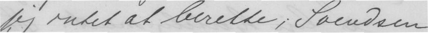jeg intet at berette; Svendsen
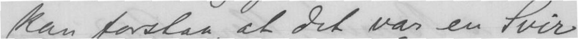kan forstaa, at det var en Svir
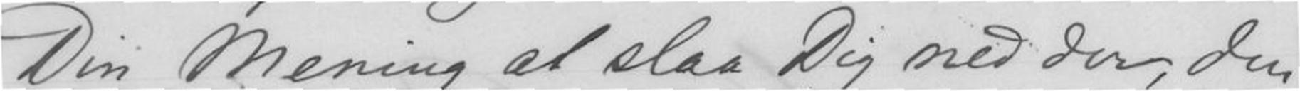Din Mening at slaa Dig ned der, den
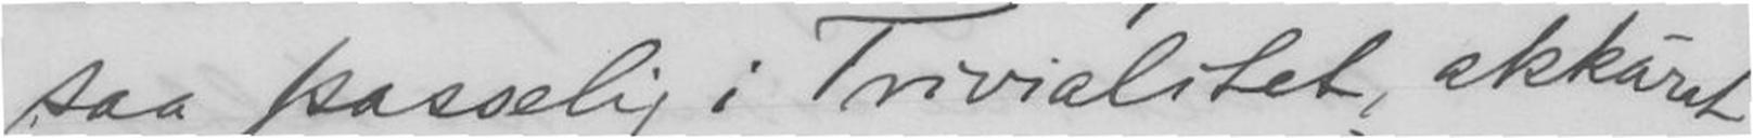saa passelig i Trivilitet, akkurat
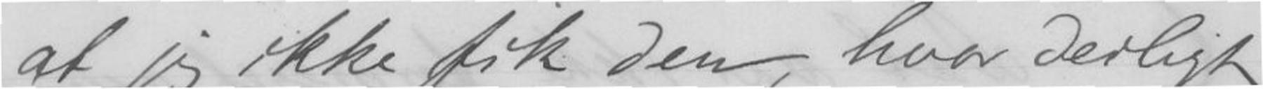at jeg ikke fik den, hvor deiligt
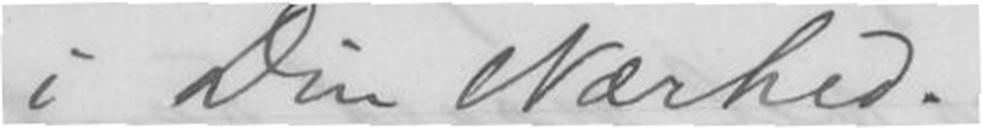i Din Nærhed.
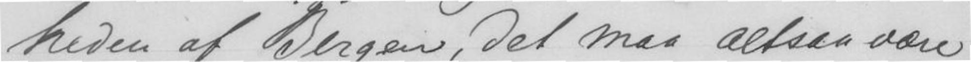heden af Bergen, det maa altsaa være
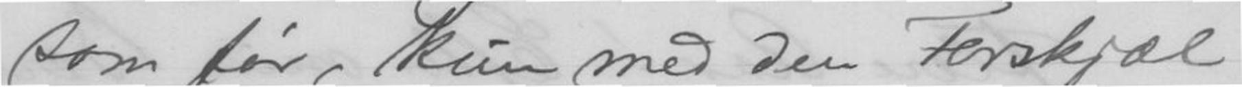som før, kun med den Forskjæl
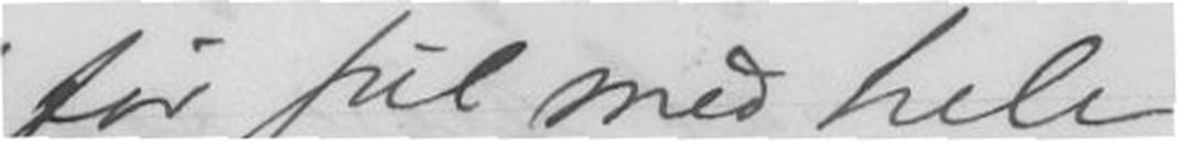før Jul med hele
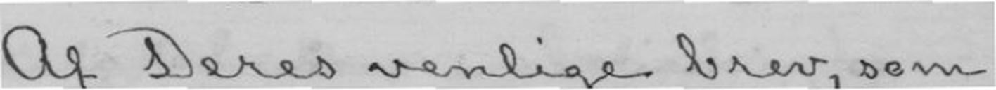Af Deres venlige brev, som
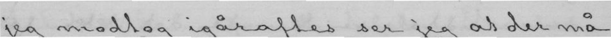jeg modtog igåraftes ser jeg at der må
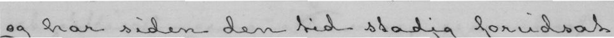og har siden den tid stadig forudsat
